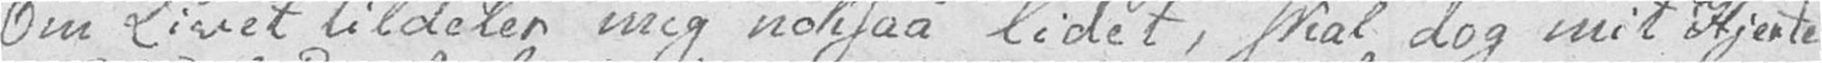Om Livet tildeler mig noksaa lidet, skal dog mit Hjerte
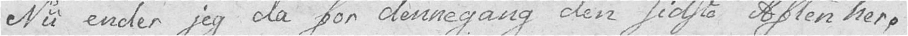Nu ender jeg da for dennegang den sidste Aften her.
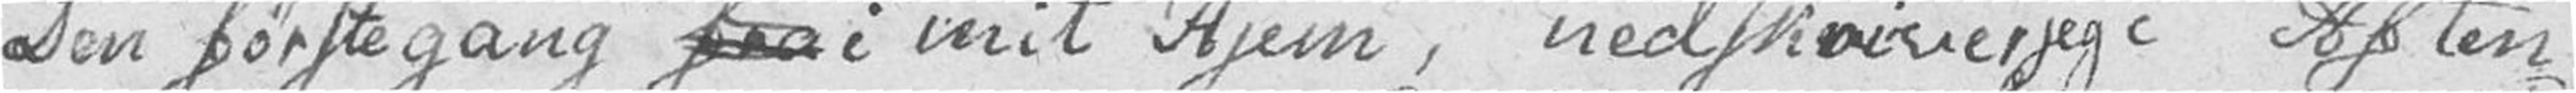Den første gang fra i mit Hjem, nedskriver jeg i Aften
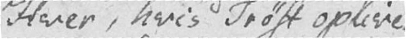Hver, hvis Trøst opliver
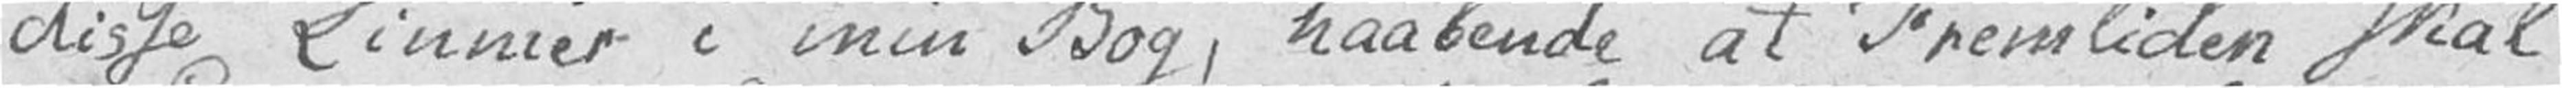disse Linnier i min Bog, haabende at Fremtiden skal
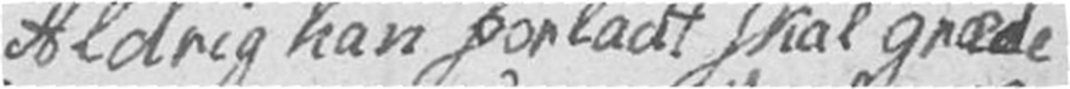Aldrig han forladt skal græde
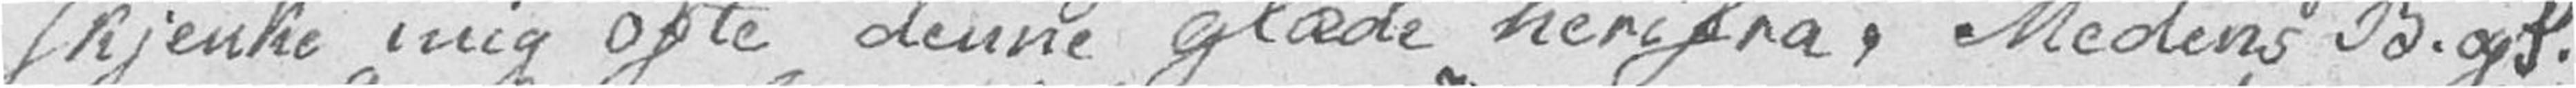skjenke mig ofte denne glæde herifra, Medens B. og S.
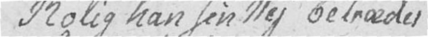Rolig han sin Vej betræder
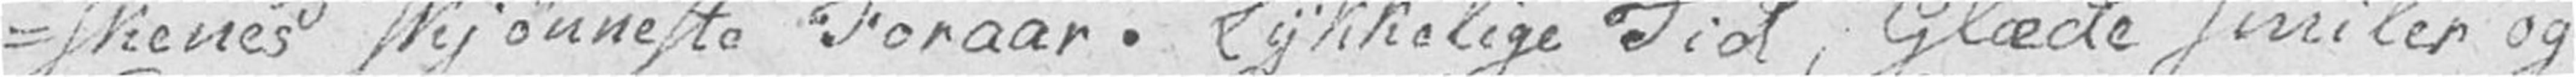-skenes skjønneste Foraar. Lykkelige Tid, Glæde smiler og
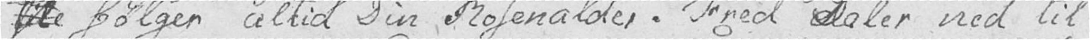følger altid Din Rosenalder. Fred Daler ned til
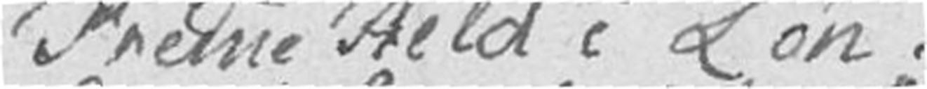Fremme Held i Løn!
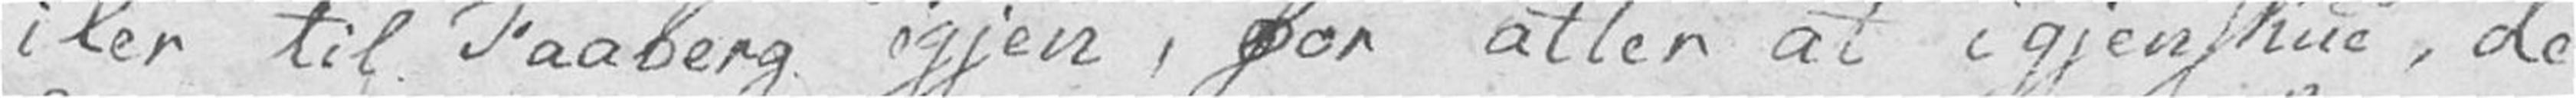iler til Faaberg igjen, for atter at igjenskue, de
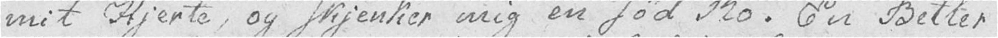mit Hjerte, og skjenker mig en sød Ro. En Betler
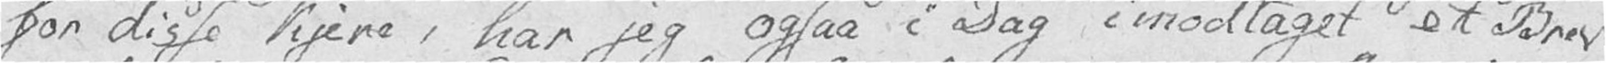for disse kjere, har jeg ogsaa i Dag imodtaget et Brev
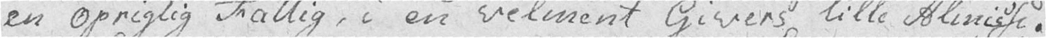en oprigtig Fattig, i en velment Givers lille Almisse.
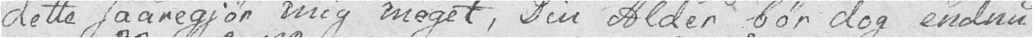dette saaregjør mig meget, Din Alder bør dog endnu
